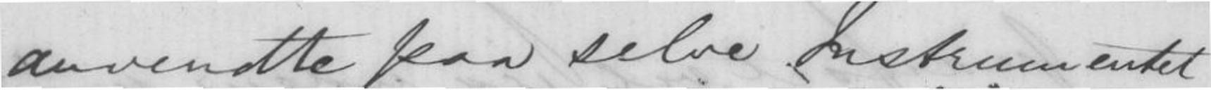anvendte paa selve Instrumentet
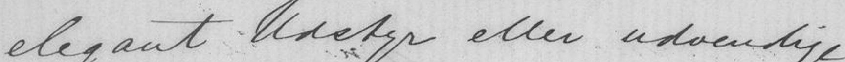elegant Udstyr eller udvendige
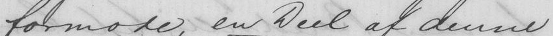formode, en Deel af denne
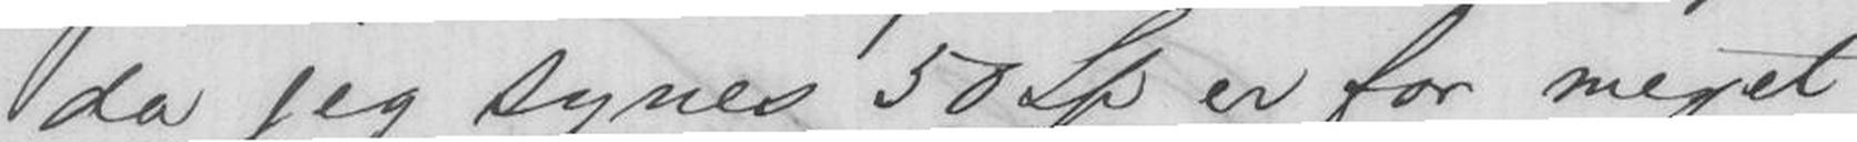da jeg synes 50 Sp er for meget
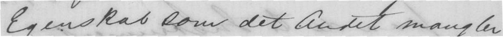Egenskab som det Andet mangler,
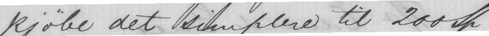kjøbe det simplere til 200 Sp
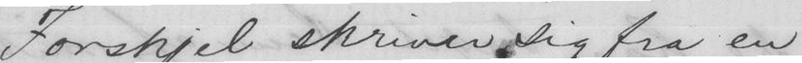Forskjel skriver sig fra en
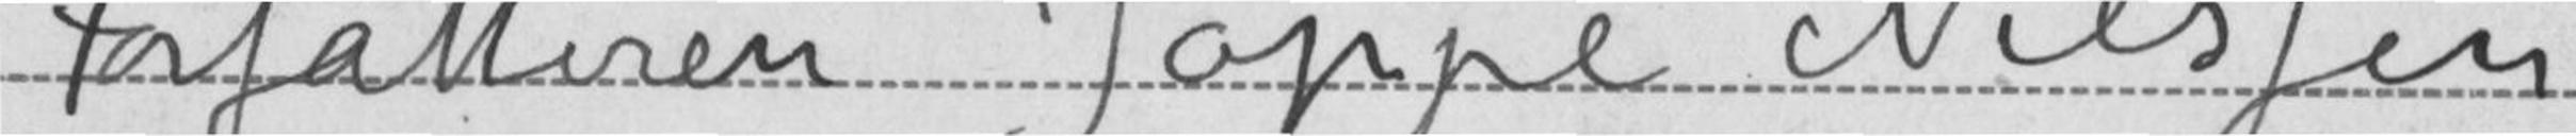Forfatteren Jappe Nilssen
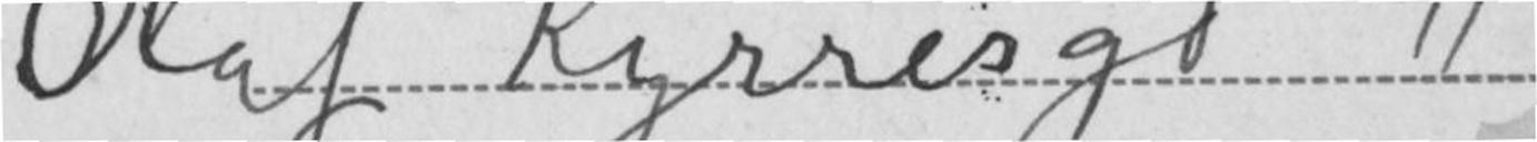Olaf Kyrresgd 11
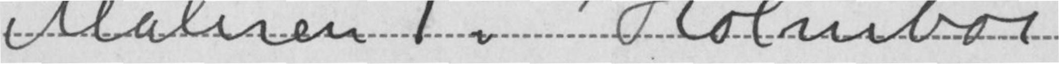Maleren T. Holmboe
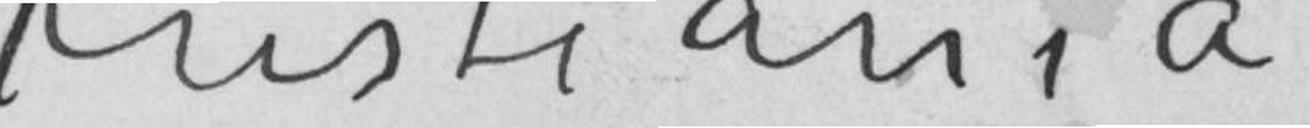Kristiania
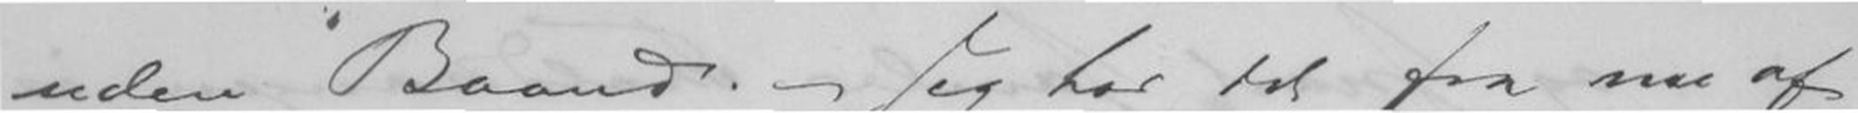uden Baand. - Jeg har det fra nu af
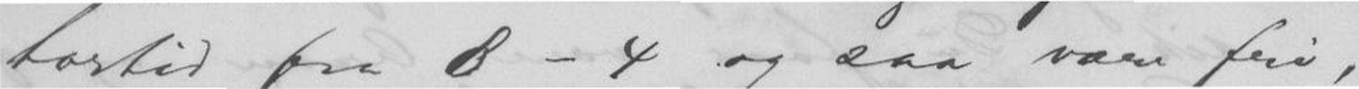tortid fra 8 - 4 og saa være
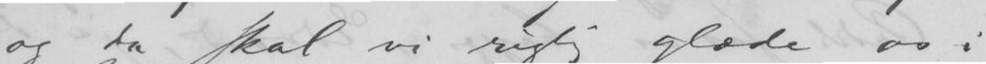fri, og da skal vi rigtig glæde os i
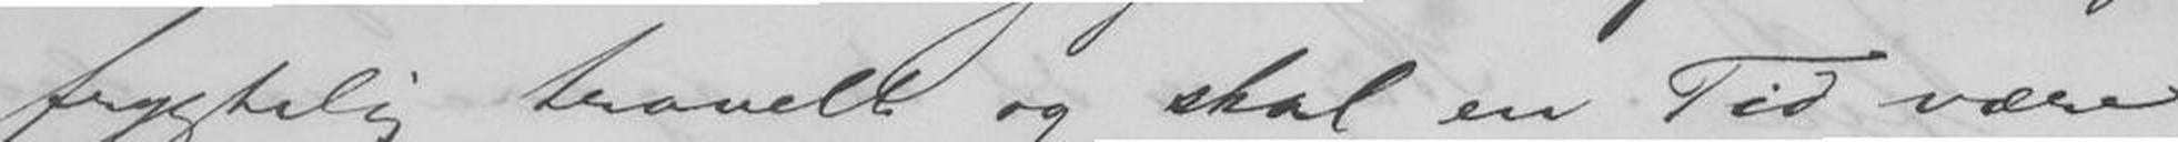fryktelig travelt og skal en Tid være
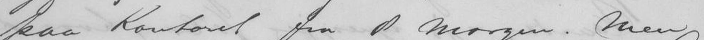paa Kontoret fra 8 Morgen. Men
